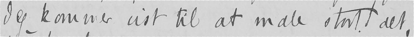Jeg kommer vist til at male stort det,
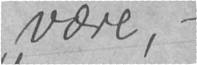være, -
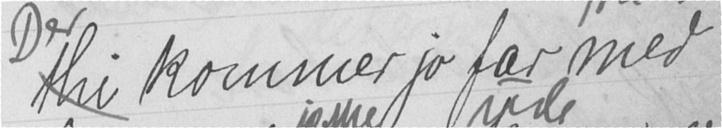Der thi kommer jo far med
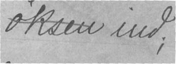øksen ind;
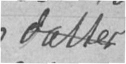datter
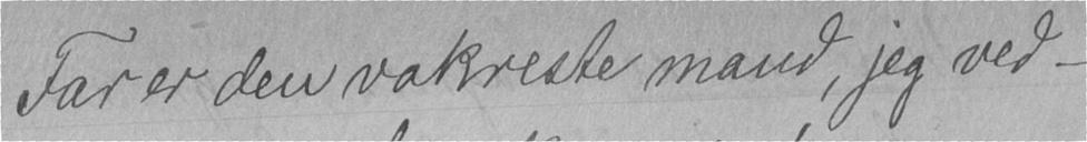Far er den vakreste mand, jeg ved -
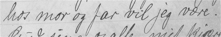hos mor og far vil jeg være.
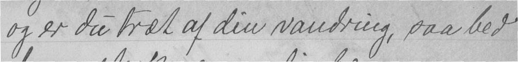og er du træt af din vandring, saa bed
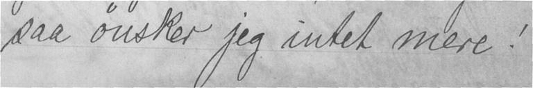saa ønsker jeg intet mere!
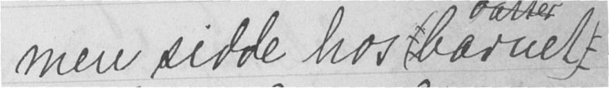men sidde hos (barnet)
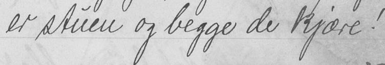er stuen og begge de kjære!
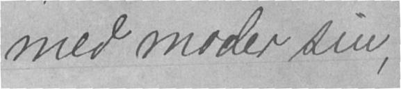med moder sin,
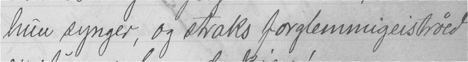hun synger, og straks forglemmigeistrøed
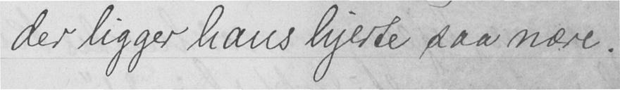der ligger hans hjerte saa nære.
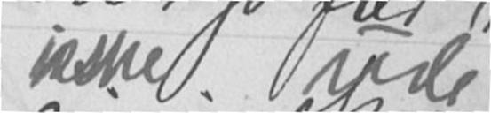ikke ude
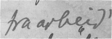fra arbeid
